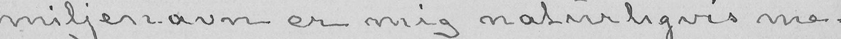miljenavn er mig naturligvis me-
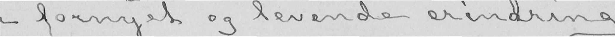i fornyet og levende erindring.
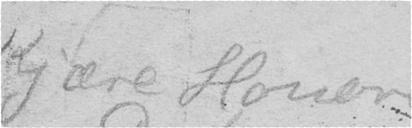Kære Honoria!
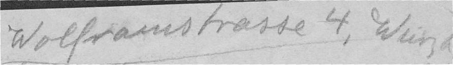Wolframstrasse 4, Win
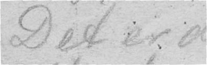Det er det værste jeg har hørt!
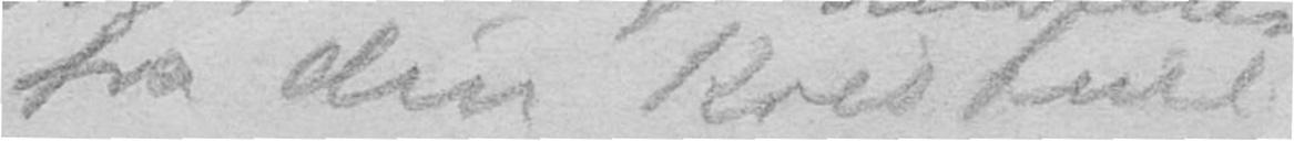fra din Kristine
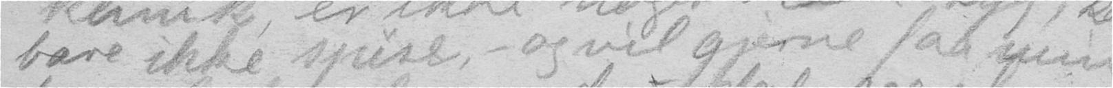bare ikke spise, - og vil gjerne faa min
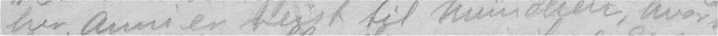her, Anni er reist til München, hvor hun
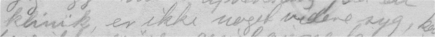klinik, er ikke noget videre syg, kan
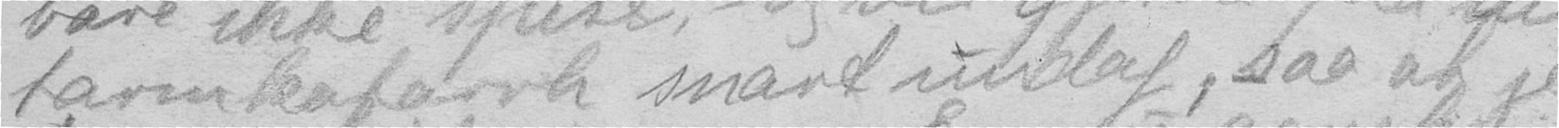tarmkatarrh snart undaf, saa at jeg
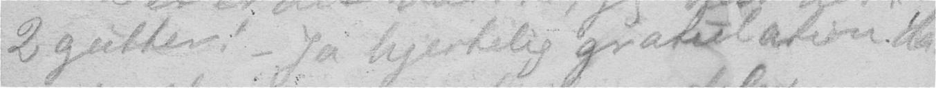2 gutter! - Ja hjertelig gratulation da!
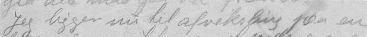Jeg ligger nu til afveksling paa en
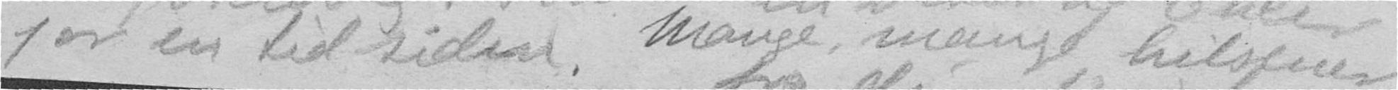for en tid siden. Mange, mange hilsener
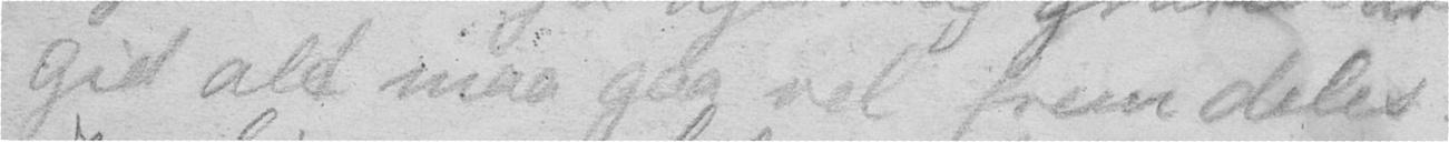gid alt maa gaa vel fremdeles. -
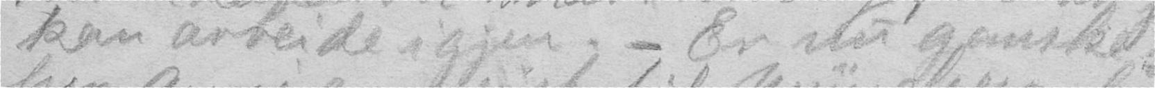kan arbeide igjen. - Er nu ganske solo
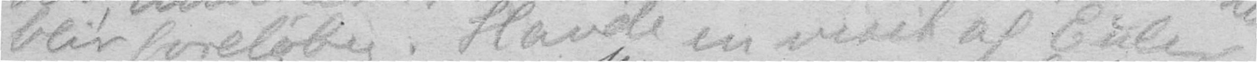blir foreløbig. Havde en visit af Euler
